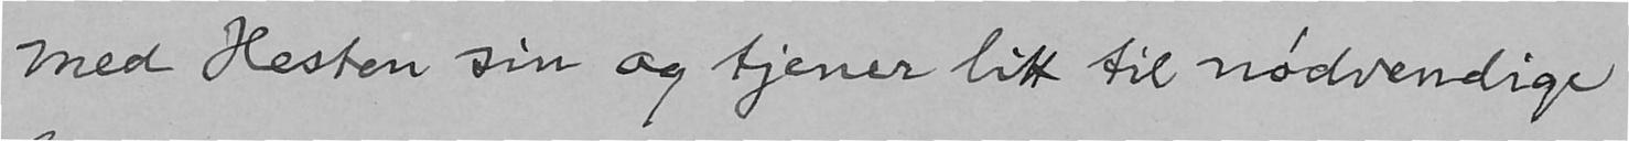med Hesten sin og tjener litt til nødvendige
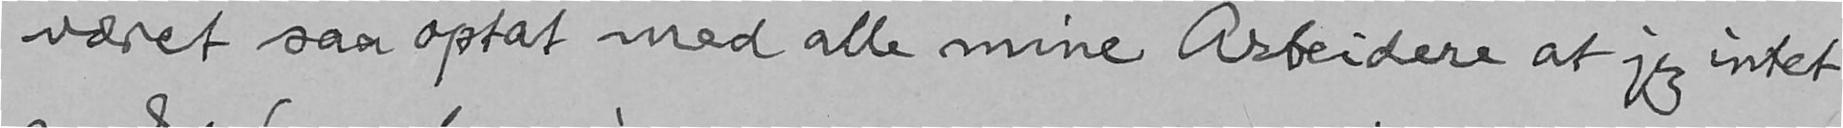været saa optat med alle mine Arbeidere at jeg intet
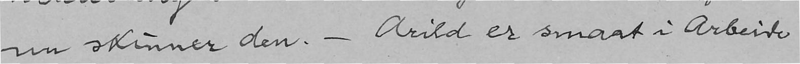nu skinner den. - Arild er smart i Arbeide
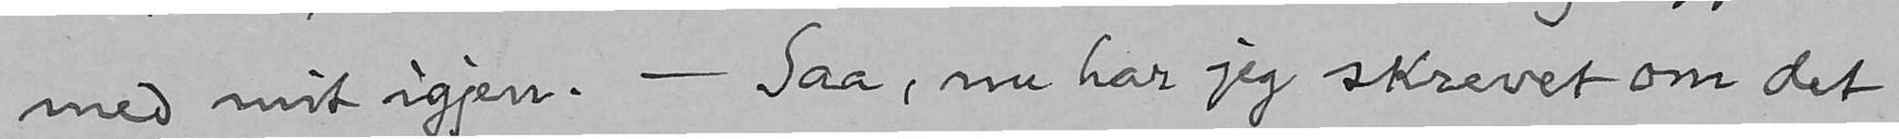med mit igjen. - Saa, nu har jeg skrevet om det
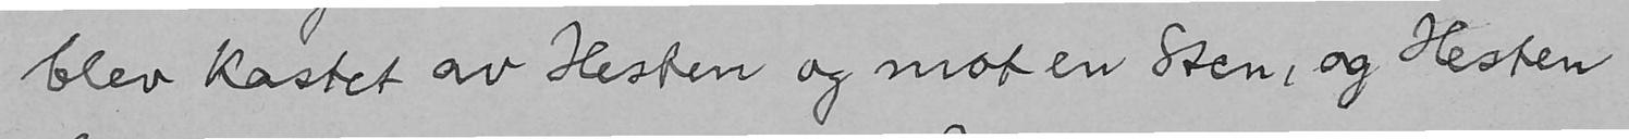blev kastet av Hesten og mot en Sten, og Hesten
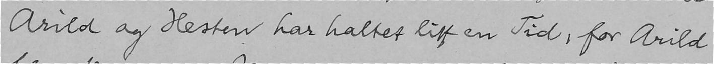Arild og Hesten har haltet litt en Tid, for Arild
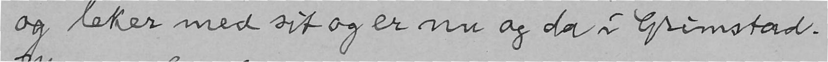og leker med sit og er nu og da i Grimstad.
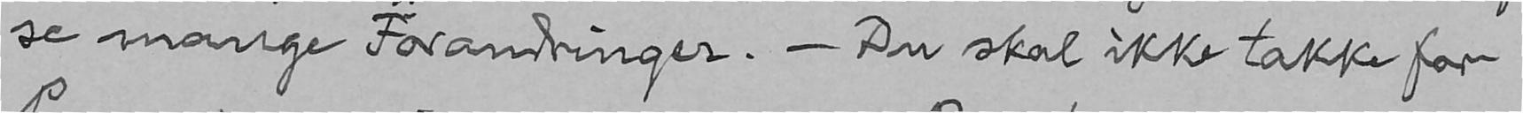se mange Forandringer. - Du skal ikke takke for
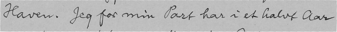Haven. Jeg for min Part har i et halvt Aar
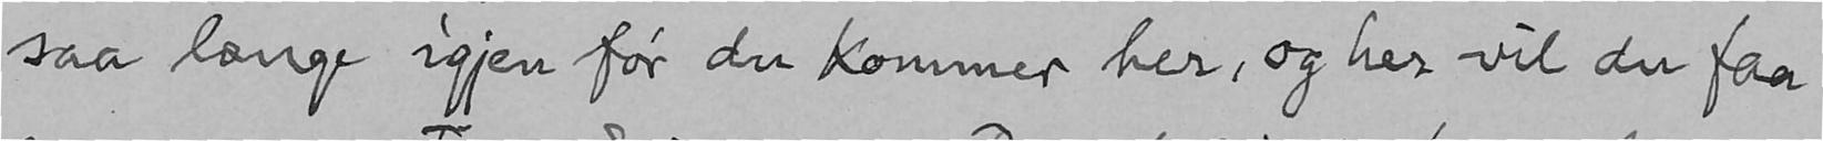saa længe igjen før du kommer her, og her vil du faa
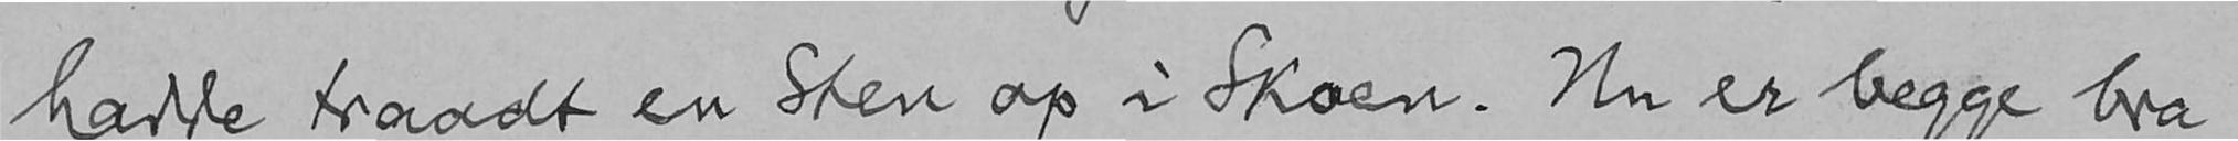hadde traadt en Sten op i Skoen. Nu er begge bra
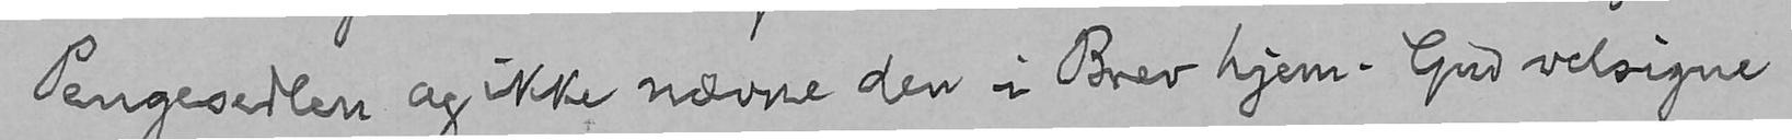Pengesedlen og ikke nævne den i Brev hjem. Gud velsigne
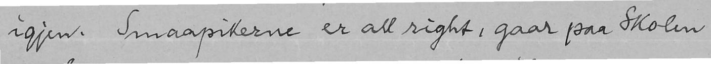igjen. Smaapikerne er all right, gaar paa Skolen
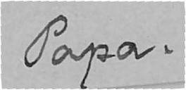Papa.
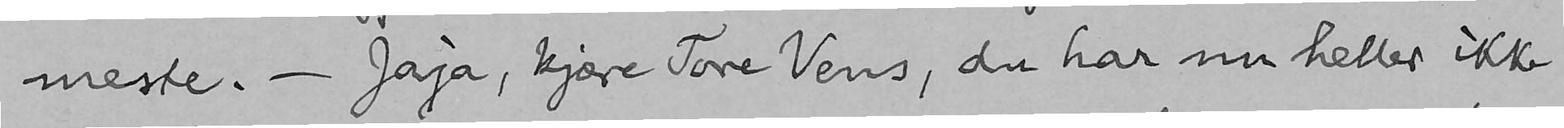meste. - Jaja, kjære Tore Vens, du har nu heller ikke
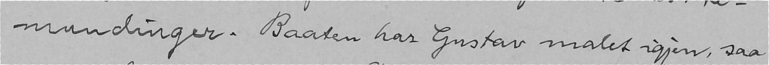mundinger. Baaten har Gustav malet igjen, saa
